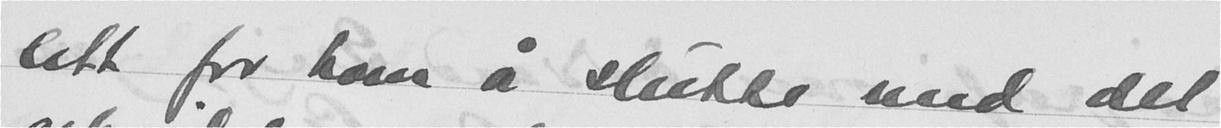lett for ham å slutte med det
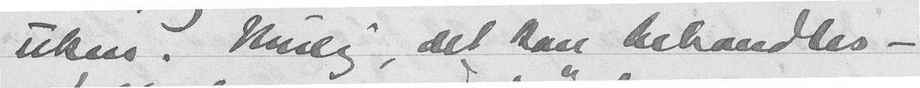uken. Mulig, det kan behandles -
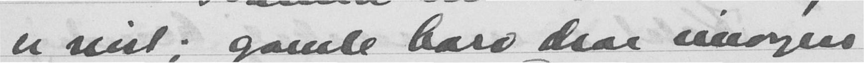er reist; gamle barn drar imorgen
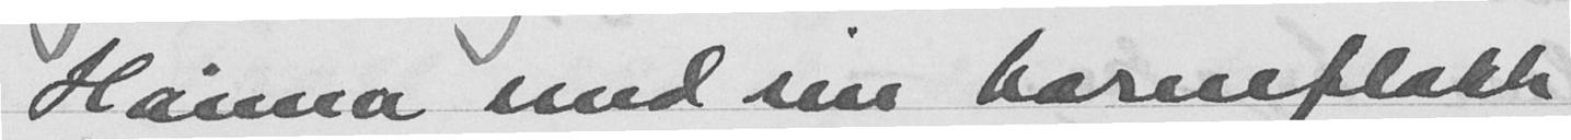Hanna med sin barneflokk
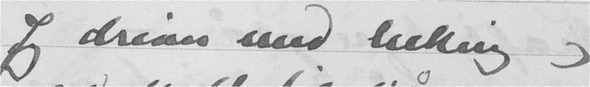Jeg driver med luking og
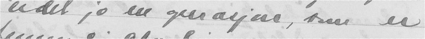er det jo en operasjon, som er
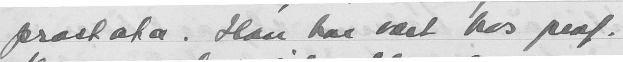prostata. Han har vært hos prof.
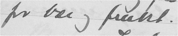for bær og frukt.
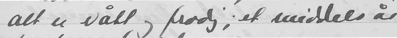alt er vått og frodig; et middels år
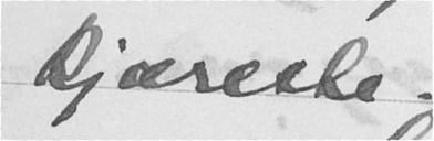kjæreste.
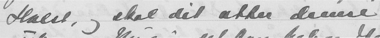Holst, og skal dit efter denne
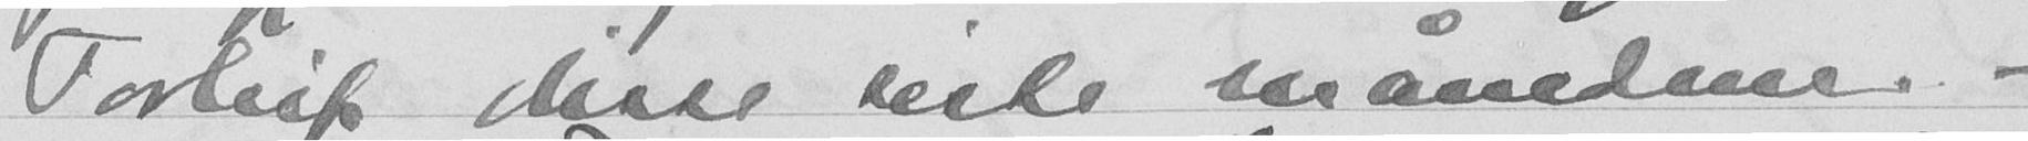Torleif disse siste månedene. -
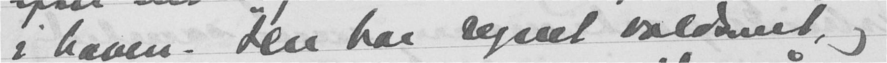i haven. Her har regnet voldsomt, og
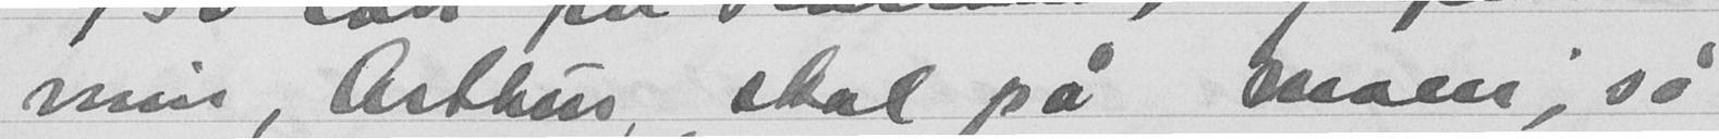min, Asthus, skal på maen, så
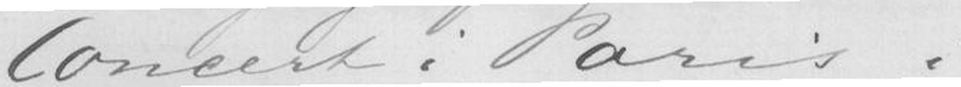Concert i Paris i
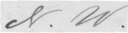N. W.
